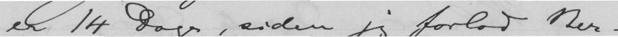er 14 Dage, siden jeg forlod Ber-
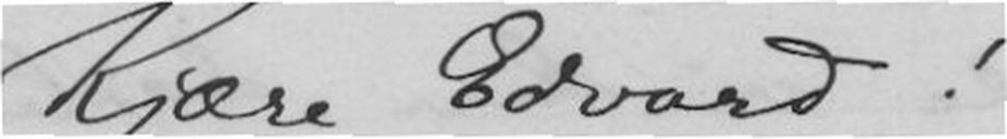Kjære Edvard!
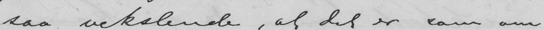saa vekslende, at det er, som om
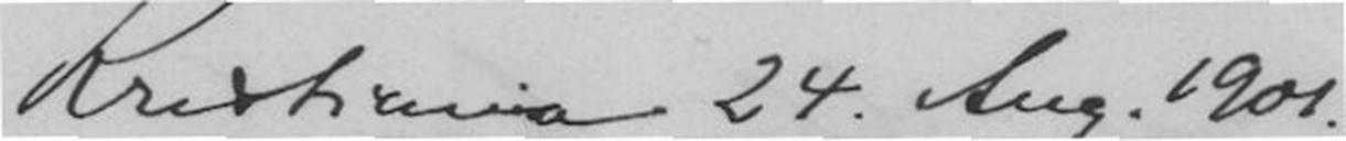Kristiania 24. Aug. 1901.
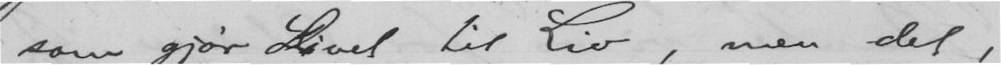som gjør Livet til Liv, men det,
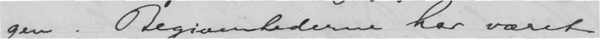gen. Begivenhederne har været
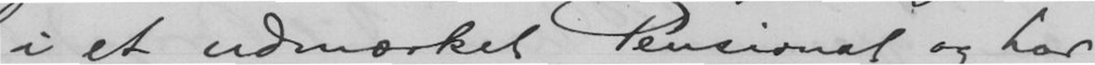i et udmærket Pensionat og har
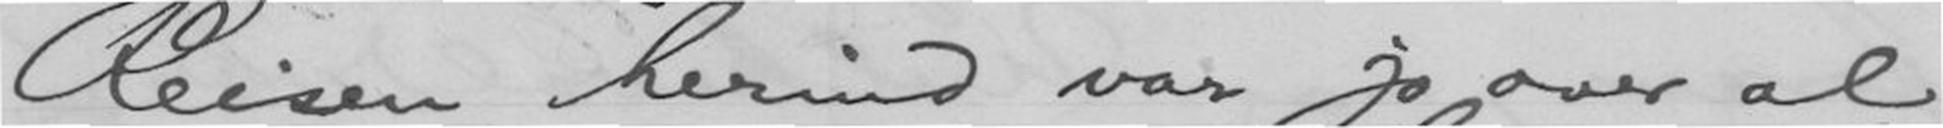Reisen herind var jeg over al
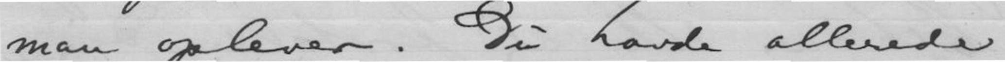man oplever. Du havde allerede
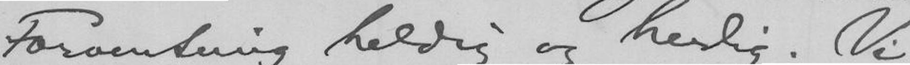Forventning heldig og herlig. Vi
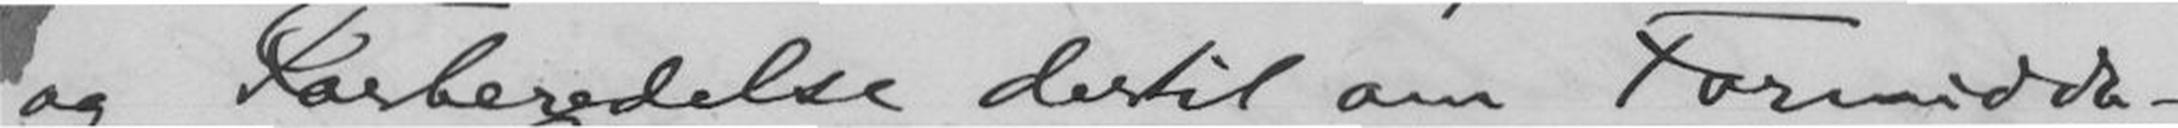og Forberedelse dertil om Formidda-
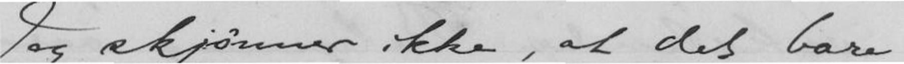Jeg skjønner ikke, at det bare
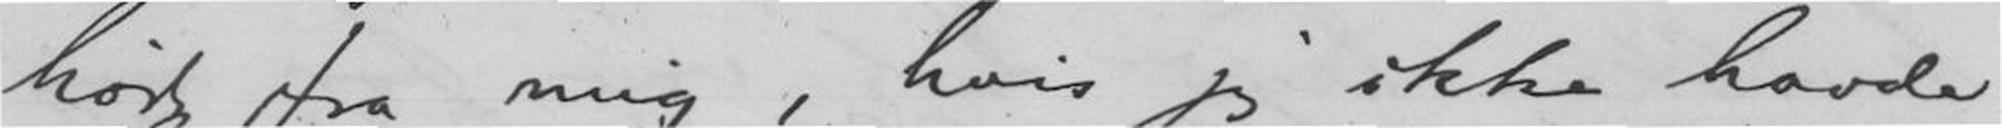hørt fra mig, hvis jeg ikke havde
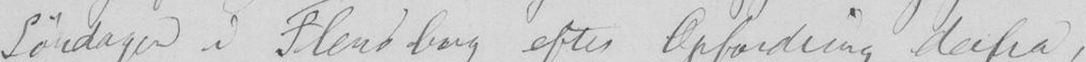Søndagen i Flensburg efter Opfordring derfra,
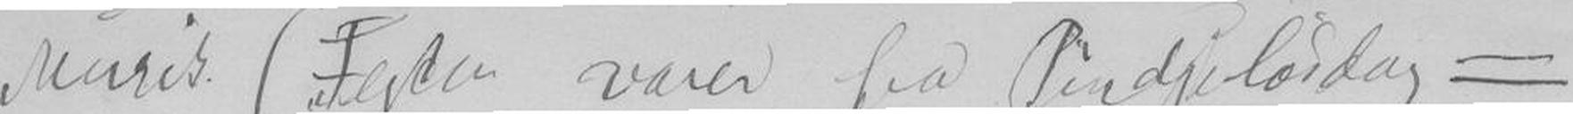Musik (Festen varer fra Pindselørdag -
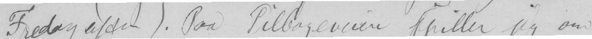Fredag aften). Paa Pilbogeveien spiller jeg om
